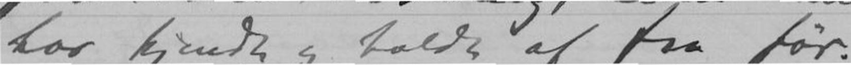har kjendt og holdt af fra før.
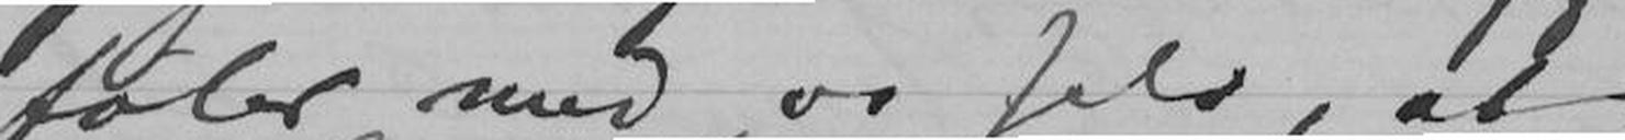føler med os selv, at
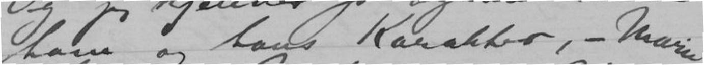ham og hans Karakter, - Marie
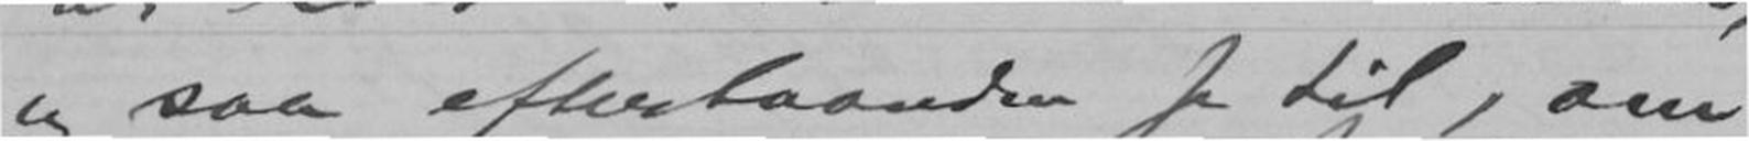og saa efterhaanden se til, om
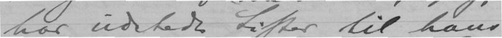har udstedt Lister til hans
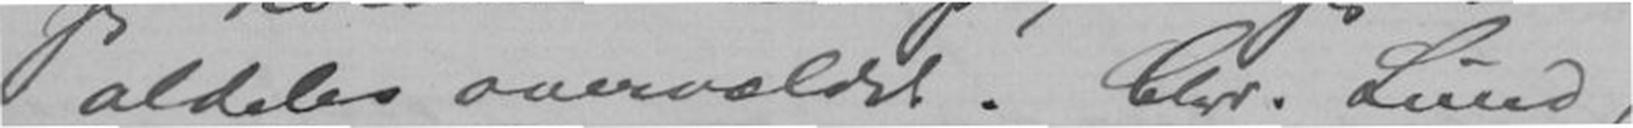aldeles overvældet. Chr. Lund,
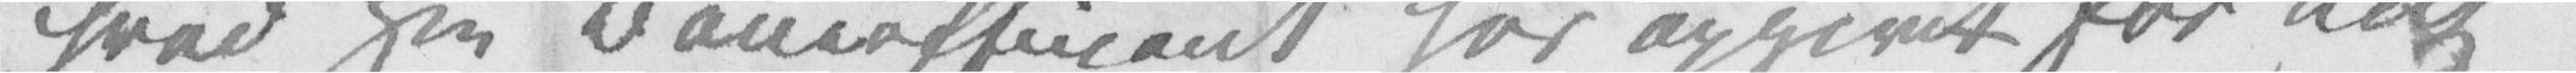hvad Hin Baneofficiant har opgivet for mig
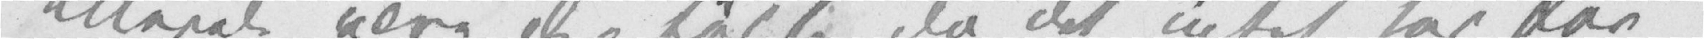allerede være der før 6 da det intet har for¬
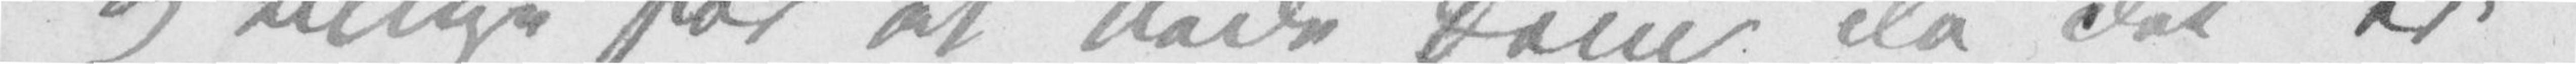og tillige for at bede Dem da det er
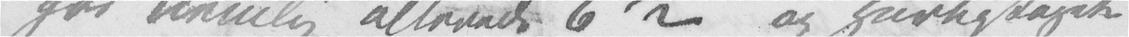går nemlig allerede 6 ½ og Hurtigtoget
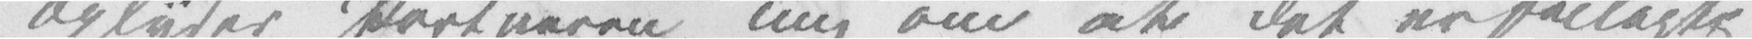oplyser Portneren mig om at det er feilagtig
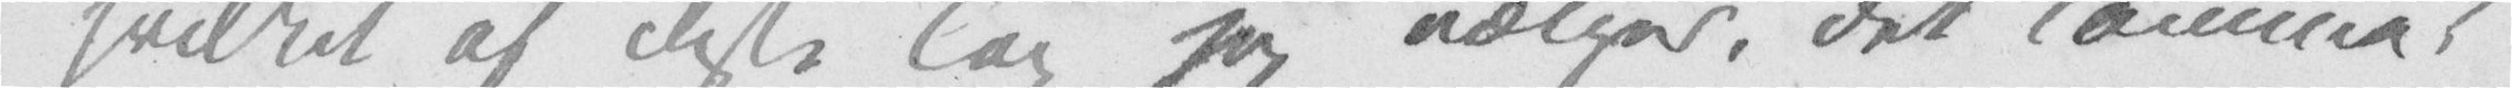hvilket af disse Tog jeg vælger, det kommer
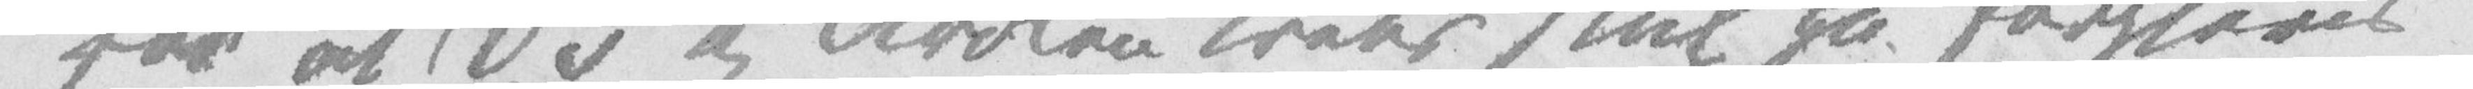for at De og Frøken Krebs skal gå forgjeves
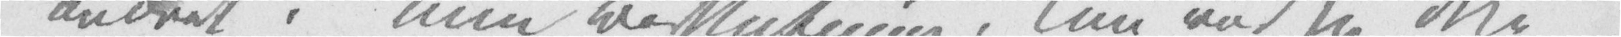andret i min Beslutning, kun ved jeg ikke
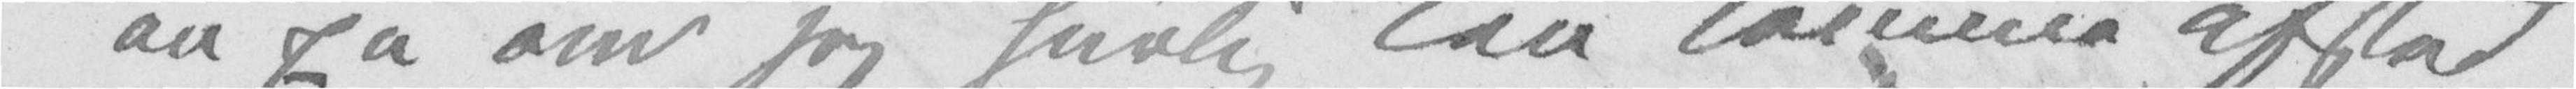an på om jeg hurtig kan komme afsted
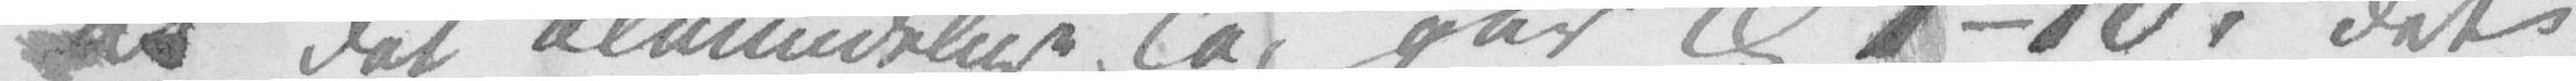at det almindelige Tog går Kl 7–10. Det
